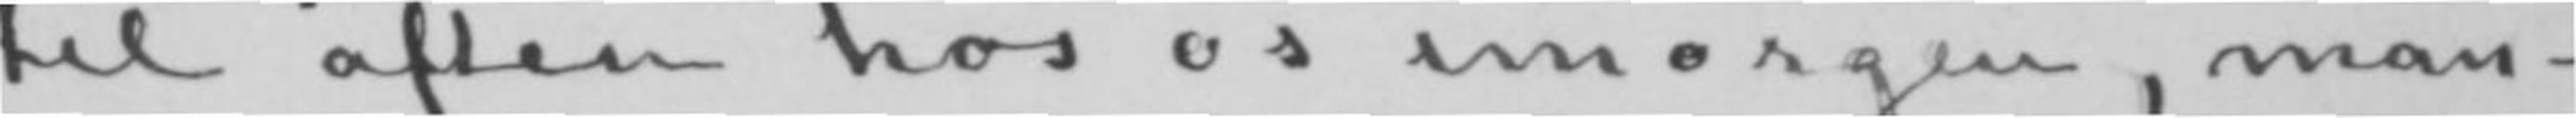til aften hos os imorgen, man-
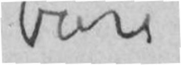bare
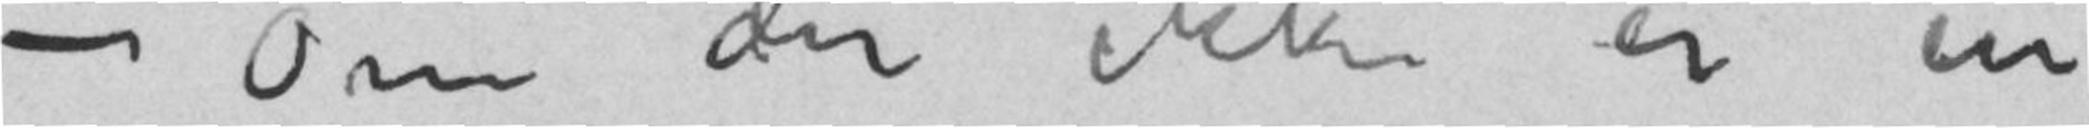– Om der ikke er en
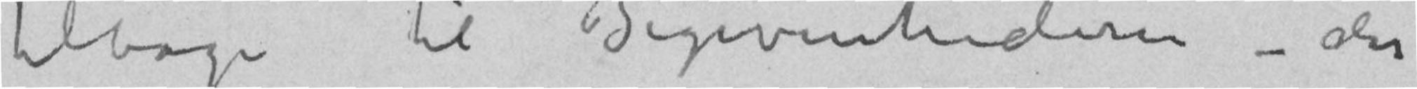tilbage til Begivenhederne – der
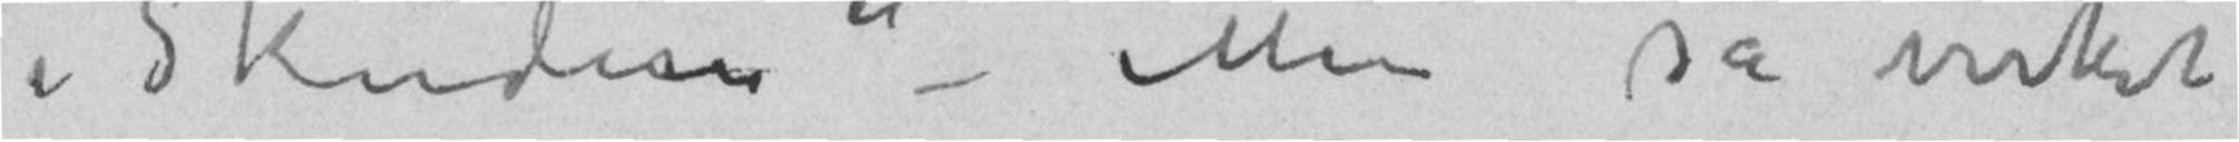i «Skuden» Men så virket
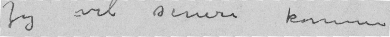Jeg vil senere komme
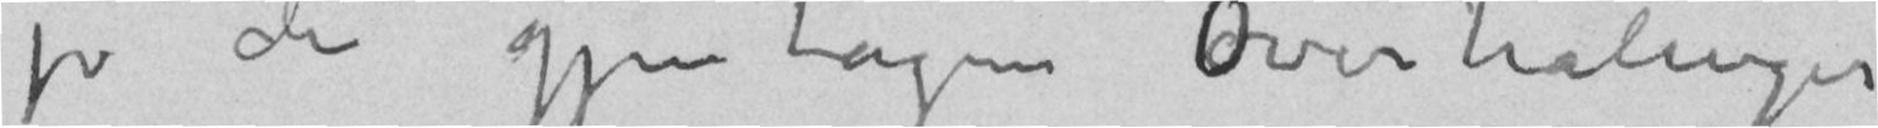jo de gjentagne Overhalninger
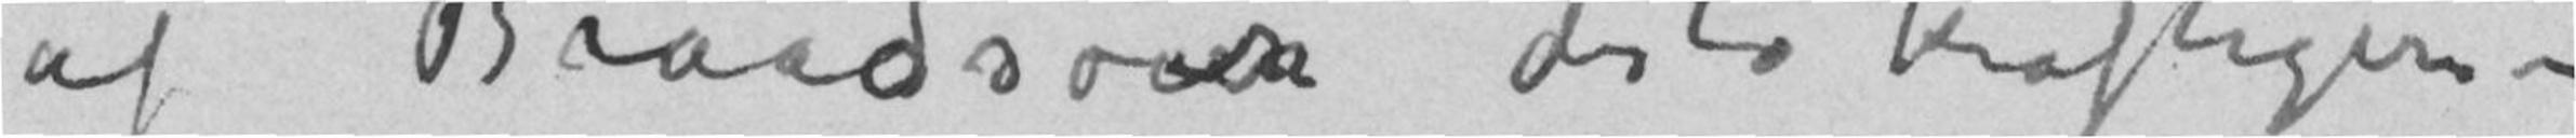af Braadsøer desto kraftigere –
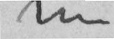nu
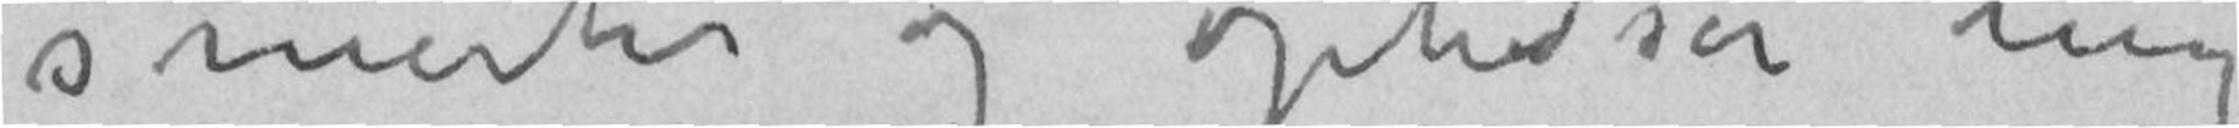smerter og ophidser mig
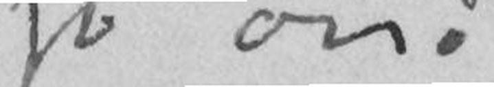jo osså
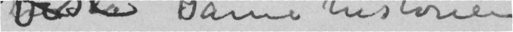vise Damehistorien
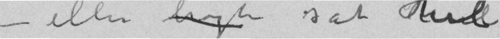– ellersat Hul
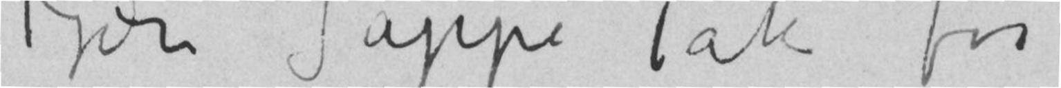Kjære Jappe! Tak for
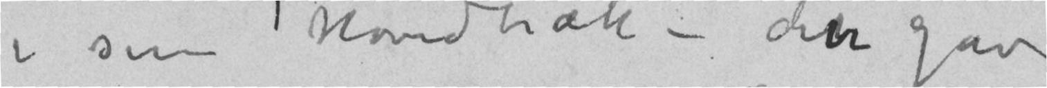i sine Hovedtræk – detn gav
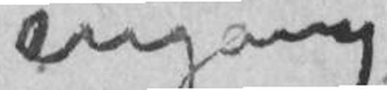engang
